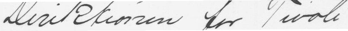Deriktøren for Tivoli
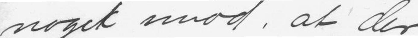noget imod, at der,
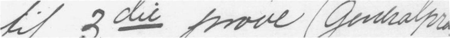til 3die prøve (Generalpr
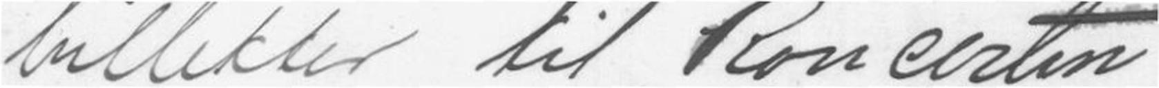billtter til koncerten
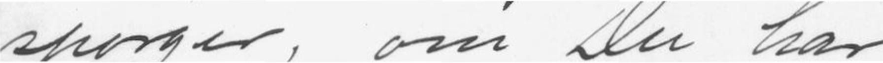spørger, om Du har
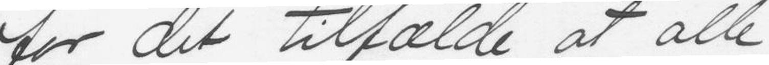for det tilfælde at alle
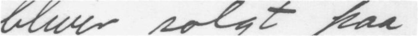bliver solgt paa
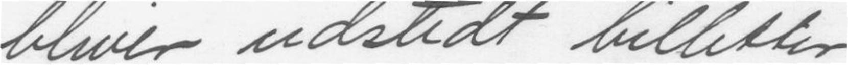bliver udstedt billetter
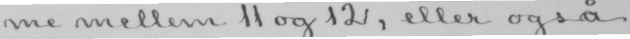me mellem 11 og 12, eller også
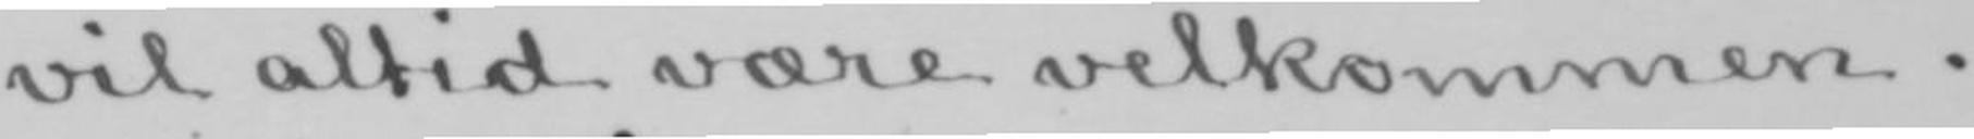vil altid være velkommen.
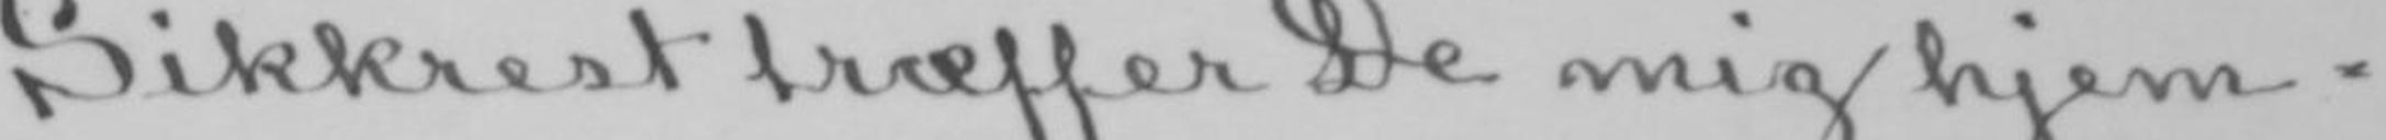Sikkrest træffer De mig hjem-
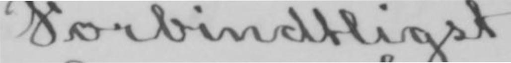Forbindtligst
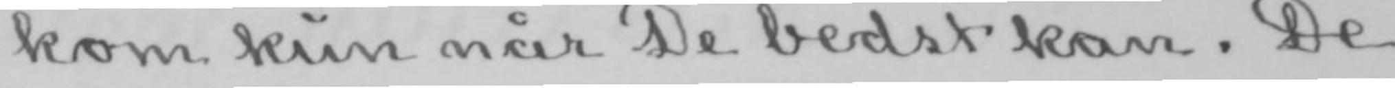kom kun når De bedst kan. De
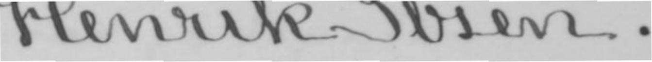Henrik Ibsen.
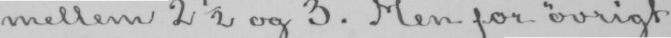mellem 2½ og 3. Men for øvrigt,
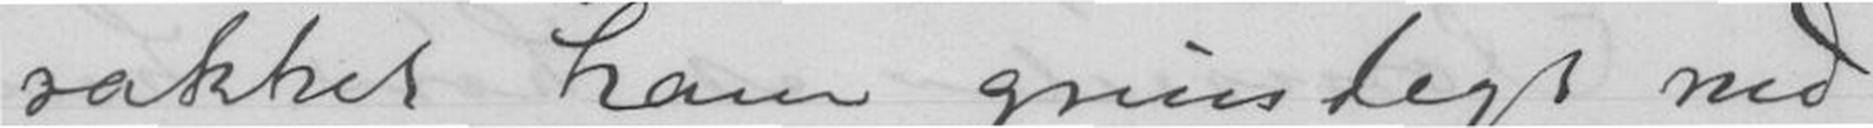rakket ham grundigt ned
